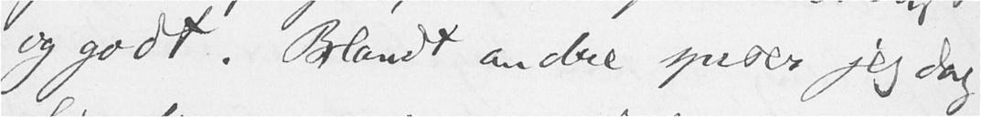og godt. Blandt andre spiser jeg dag-
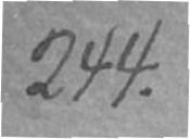244.
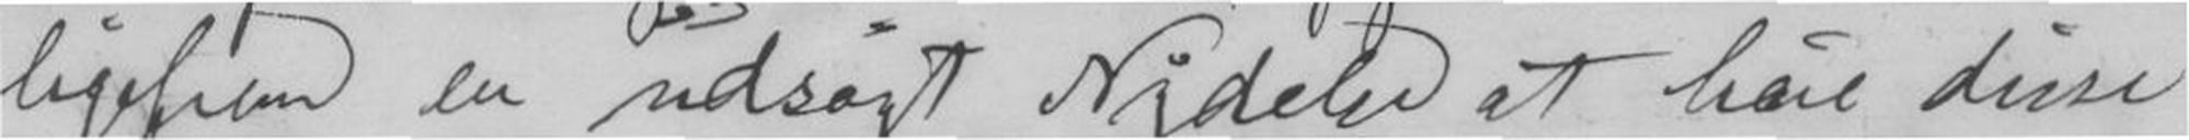ligefrem en udsøgt Nydelse at høre disse
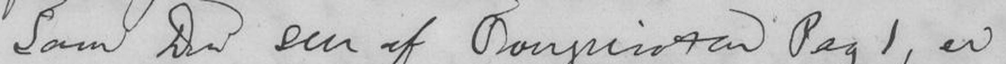Som Du seer af Overpinotar Pag 1, er
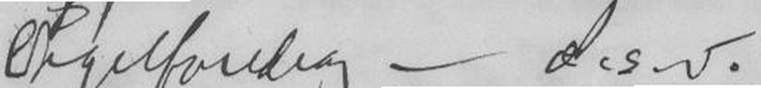Orgelforedrag - o.s.v.
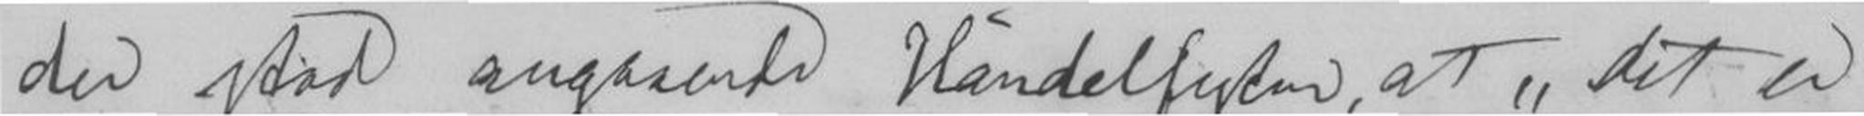der stod angaaende Händelfesten, at "det er
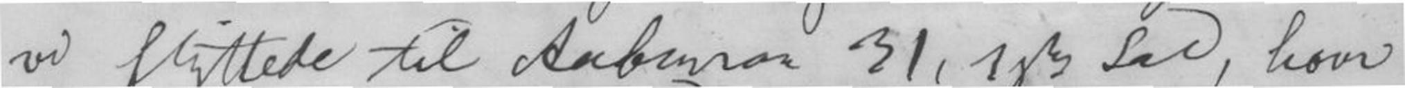vi flyttede til Aaberaa 31, 1ste Sal, have
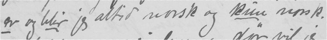er og blir jeg altid norsk og kun norsk.
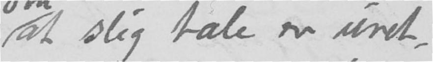at slig tale en uret-
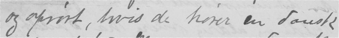og oprørt, hvis de hører en dansk
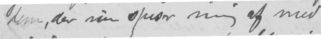dem, der nu spiser mig af med
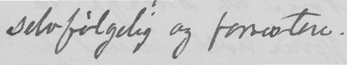selvfølgelig og forresten.
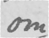om
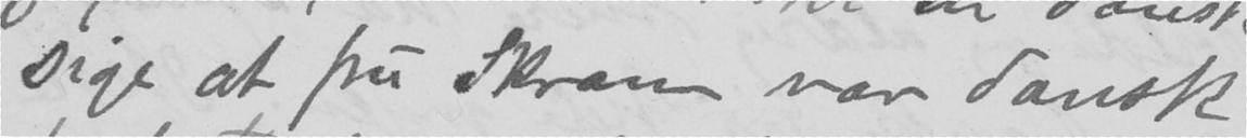sige at fru Skram var dansk
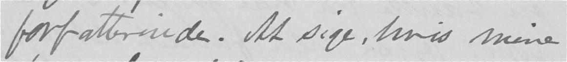forfatterinde. At sige, hvis mine
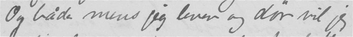Og både, mens jeg lever og dør vil jeg
